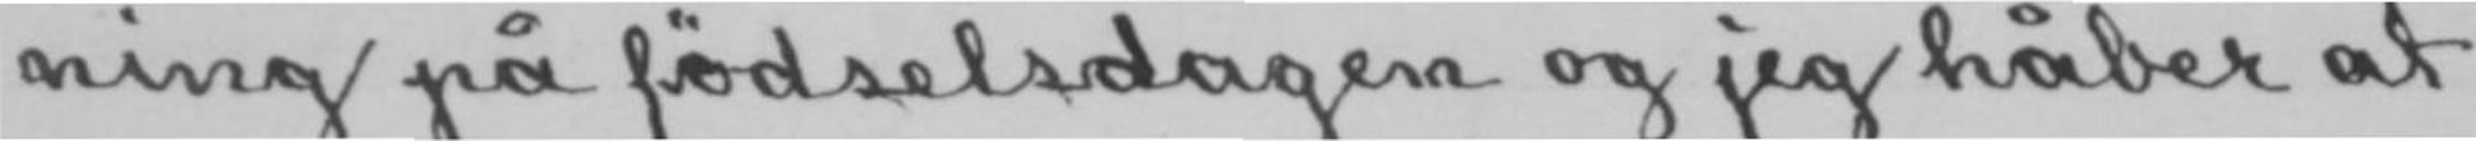ning på fødselsdagen og jeg håber at
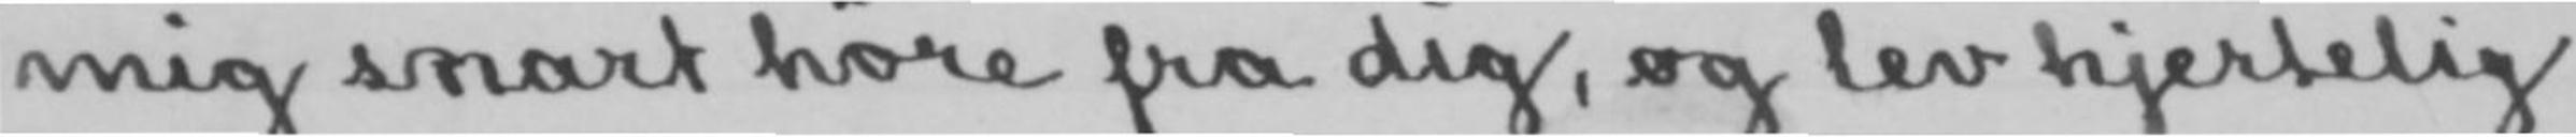mig snart høre fra dig, og lev hjertelig
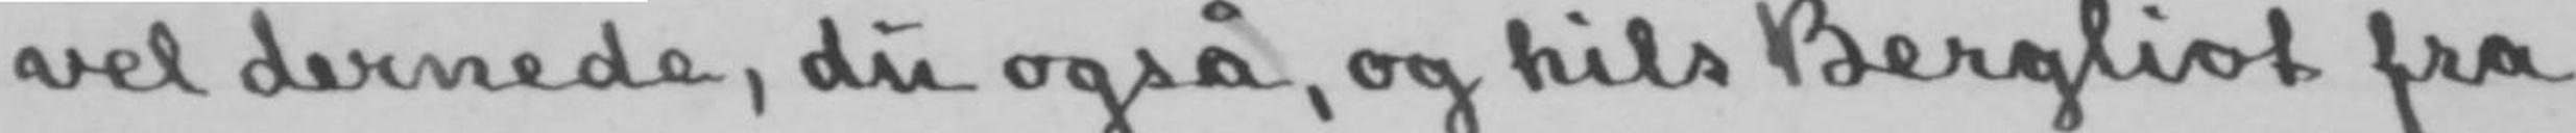vel dernede, du også, og hils Bergliot fra
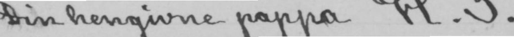Din hengivne pappa H. I.
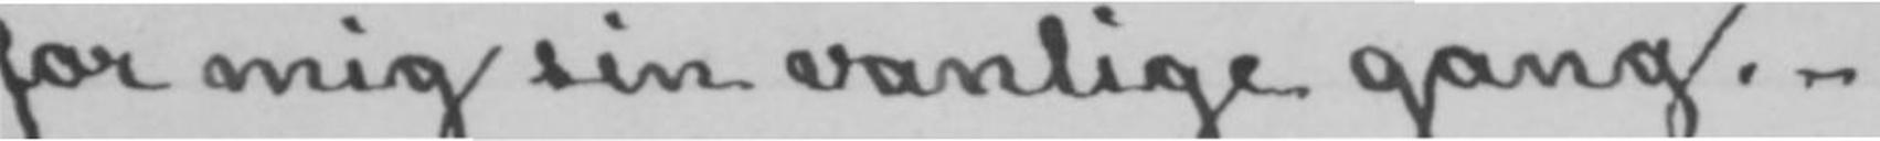for mig sin vanlige gang.-
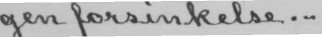gen forsinkelse.-
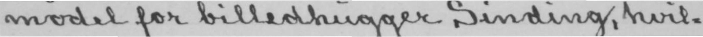model for billedhugger Sinding, hvil-
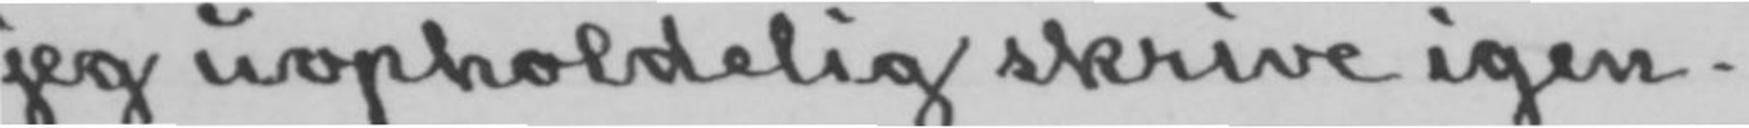jeg uopholdelig skrive igen.
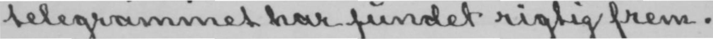telegrammet har fundet rigtig frem.
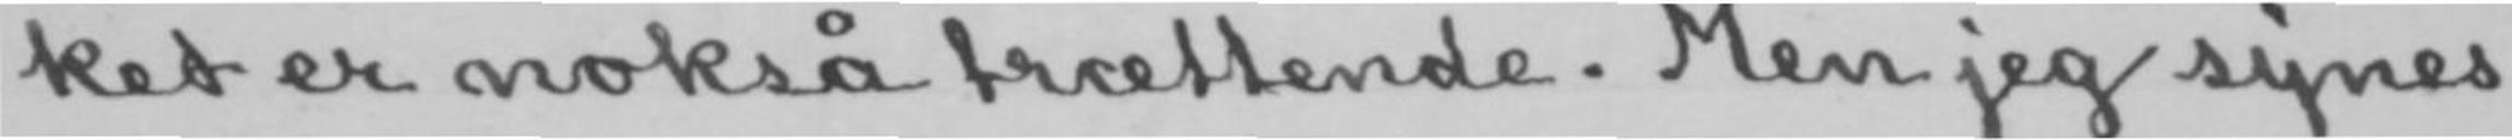ket er nokså trættende. Men jeg synes
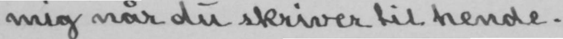mig når du skriver til hende.
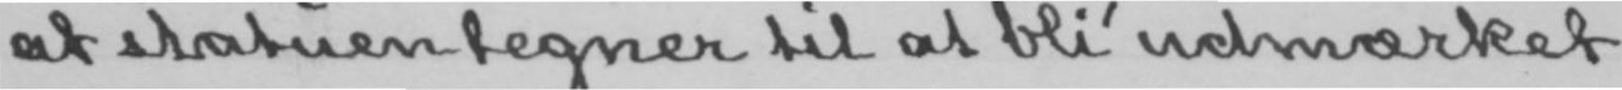at statuen tegner til at bli' udmærket
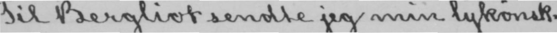Til Bergliot sendte jeg min lykønsk-
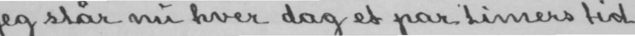Jeg står nu hver dag et par timers tid
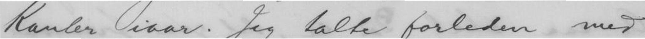Kanter iaar. Jeg talte forleden med
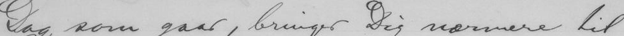Dag som gaar, bringer Dig nærmere til
